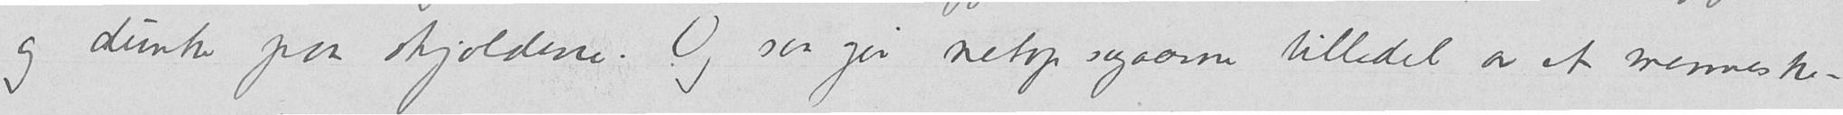og dunke paa skjoldene. Og saa gir netop sagaerne billedet av et menneske-
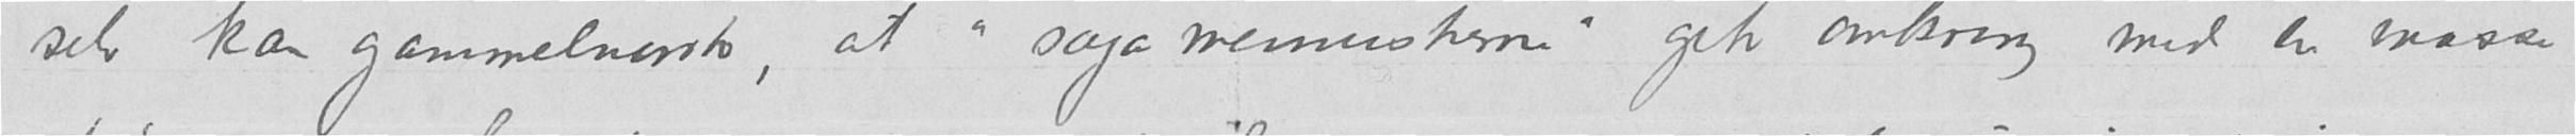selv kan gammelnorsk, at «sagamenneskerne» gik omkring med en masse
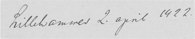Lillehammer 2. april 1922
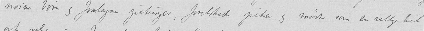naive barn og forslagne gutunger, forelskede piker og mødre som er villige til
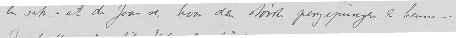en sak – at de faar se, hvor den største pengepungen er henne –.
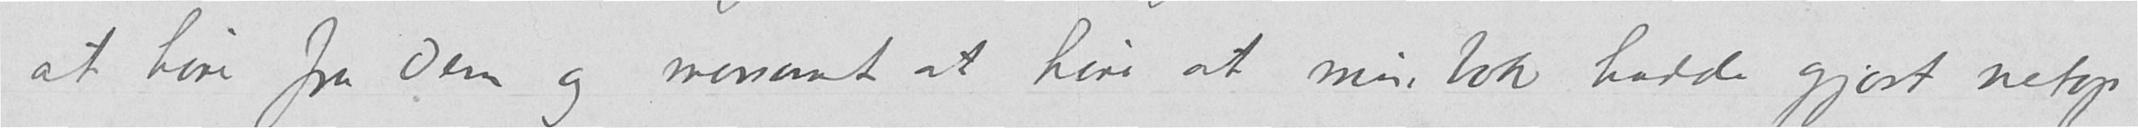at høre fra Dem og morsomt at høre at min bok hadde gjort netop
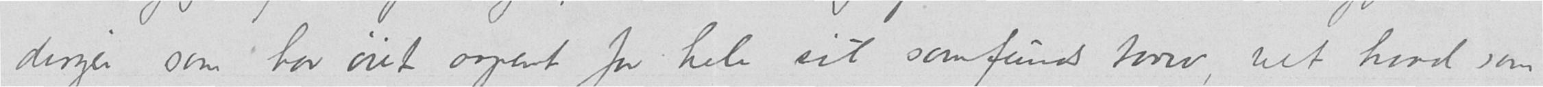dinger som har øret aapent for hele sit samfunds lover, vet hvad som
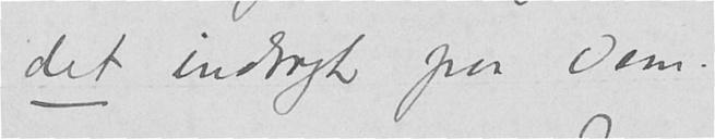det indtryk paa Dem.
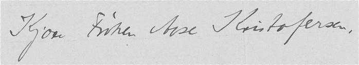Kjære Frøken Aase Kristofersen,
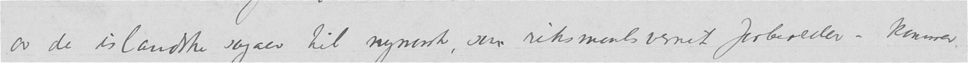av de islandske sagaer til nynorsk, som riksmaalsvernet forbereder – kommer
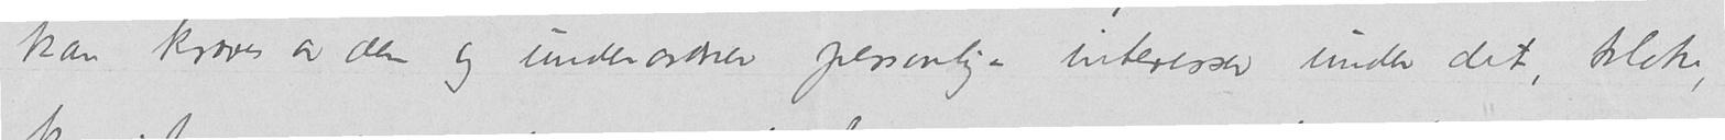kan kræves av dem og underordner personlige interesser under det, kloke,
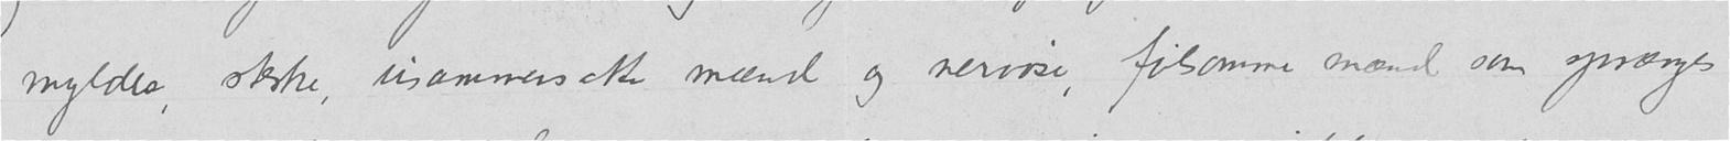mylder, sterke, usammensatte mænd og nervøse, følsomme mænd som sprænges
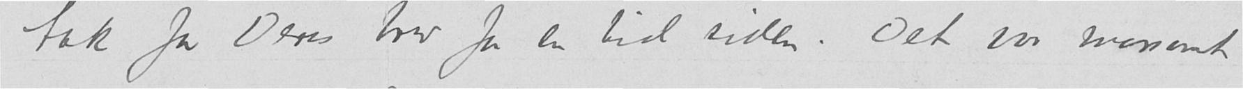tak for Deres brev for en tid siden. Det var morsomt
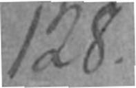128.
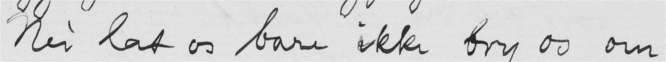Nei lat os bare ikke bry os om
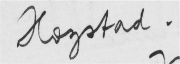Hægstad.
